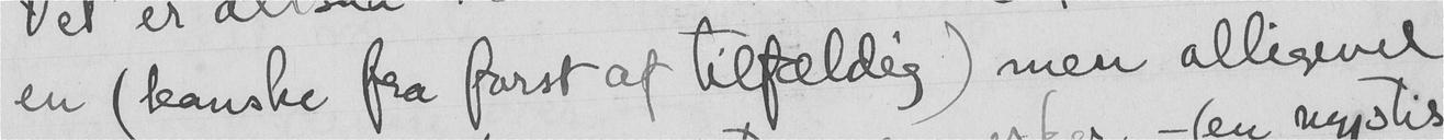en (kanske fra først af tilfældig) men alligeved
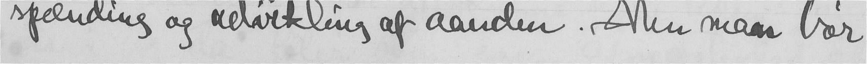spænding og udvikling af aanden. Men maa bør
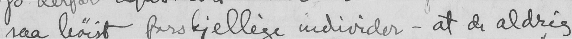saa høist forskjellige individer - at de aldrig
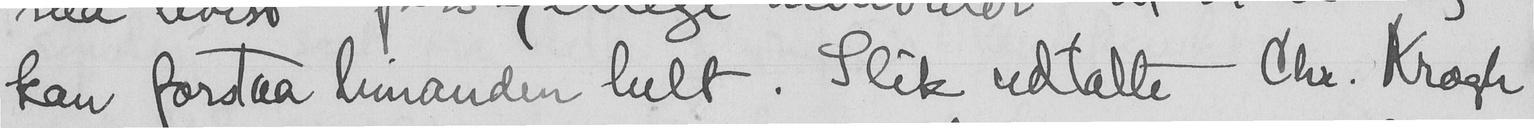kan forstaa hinanden helt. Slik udtalte Chr. Krogh
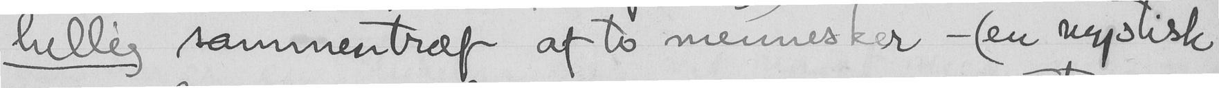hellig sammentræf af to mennesker - (en mystisk
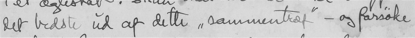det bedste ud af dette "sammentræf" - og forsøke
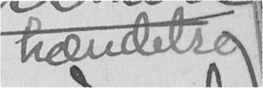hændelse
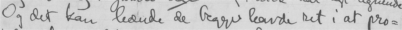Og det kan hænde de begge havde ret i at pro-
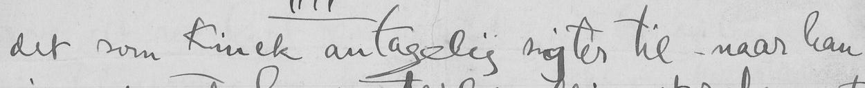det som Kinck antagelig sigter til - naar han
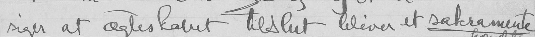siger at ægteskabet tilslut bliver et sakramente
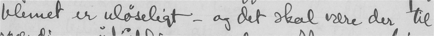blemet er uløseligt og det skal være der til
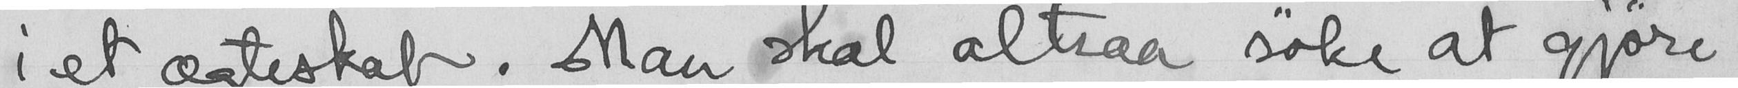i et ægteskab. Man skal altsaa søke at gjøre
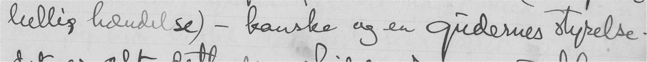hellig hændelse) - kanske og en gudernes styrelse.
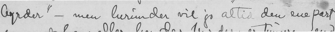byrder" - men herunder vil jo altid den ene part
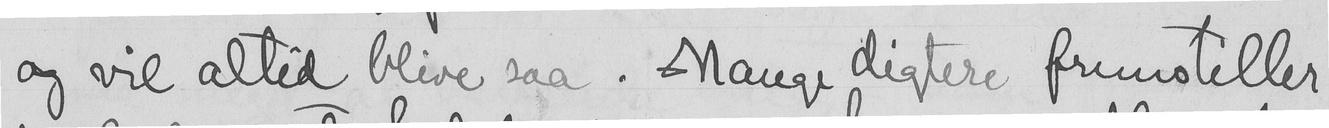og vil altid blive saa! Mange digtere fremstiller
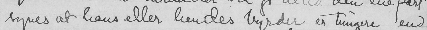synes at hans eller hendes byrder er tungere end
